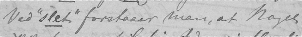Ved "slet" forstaaer man, at Noget
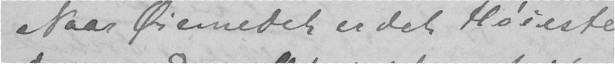Naar Øiemedet er det Høieste,
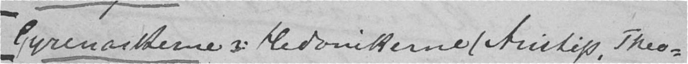[Cyrenæikerne i Hedonikerne (Aristip, Theo-
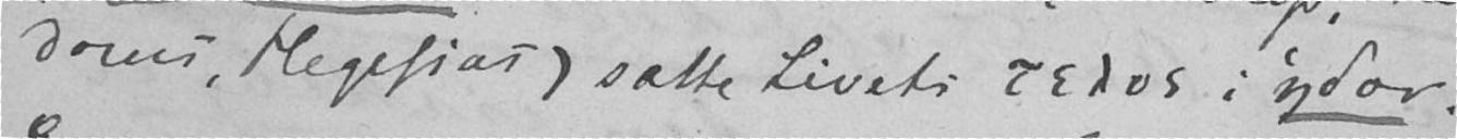dorus, Hegesias) satte Livets Telos i yder.
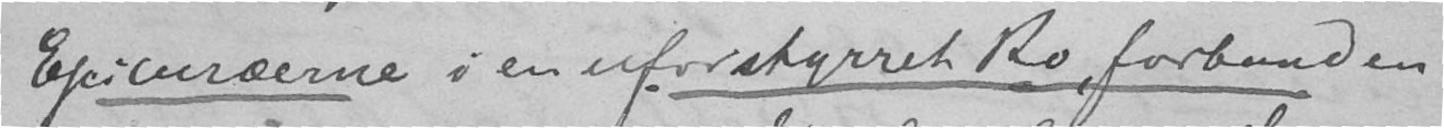Epicureerne i en uforstyrret Ro forbunden
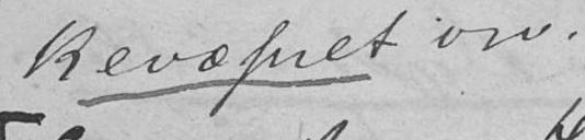kevæsenet osv.
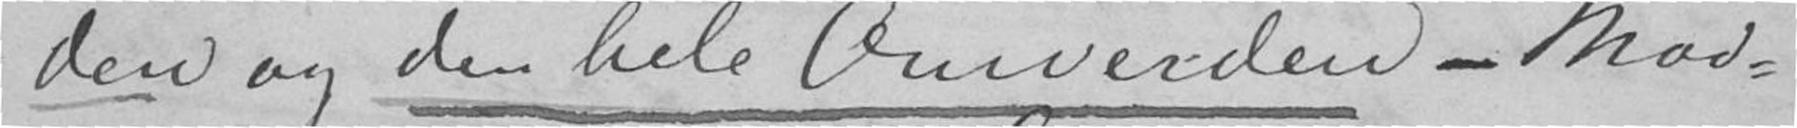den og den hele Omverden - Mod-
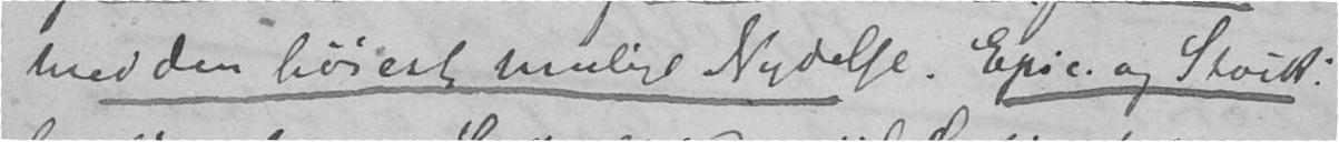med den høiest mulige Nydelse. Epic og Stoik.
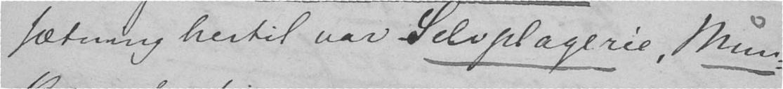sætning hertil var Selvplagerie, Mun-
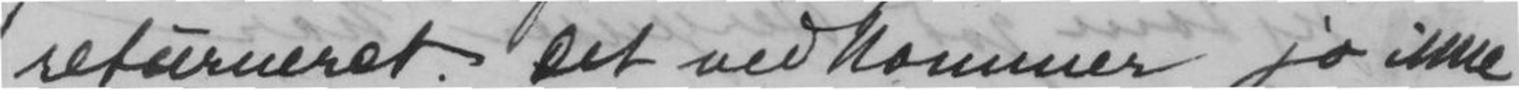retuneret. Det vedkommer jo ikke
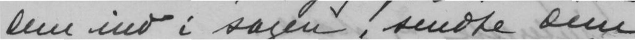Dem ind i sagen, sendte Dem
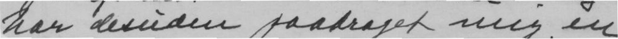har desuden paadraget mig en
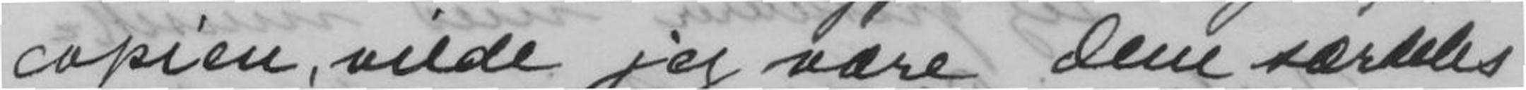copien, vilde jag være dem særdeles
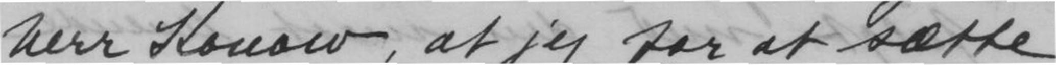herr Konow, at jeg for at sætte
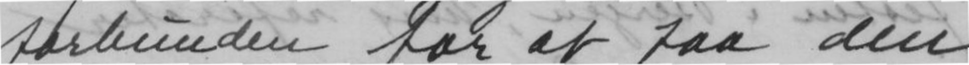forbunden for at faa den
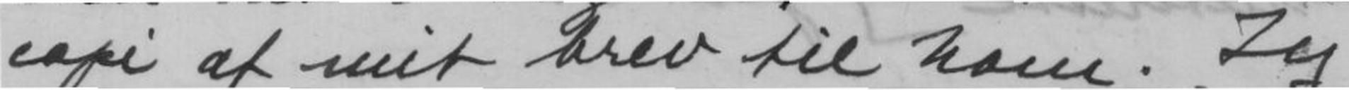copi af mit brev til ham. Jeg
